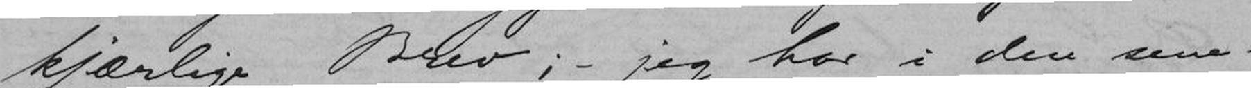kjærlige Brev; - jeg har i den sene-
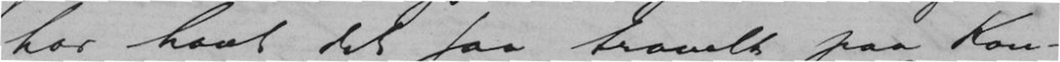har havt det saa travelt paa Kon-
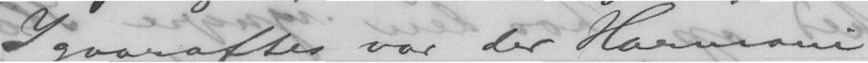Igaaraftes var der Harmoni-
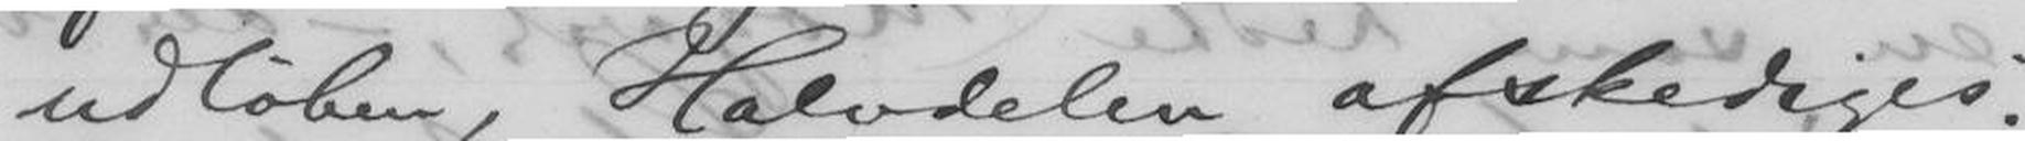udløben, Halvdelen afskediges.
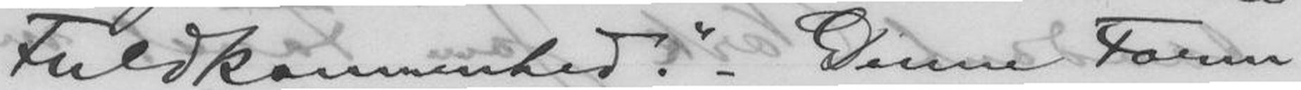Fuldkommenhed." - Denne Form
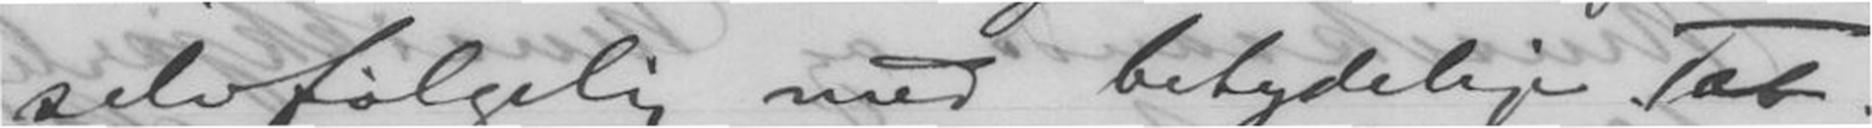selvfølgelig med betydelige Tab.
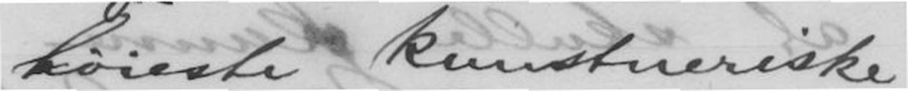høieste kunstneriske
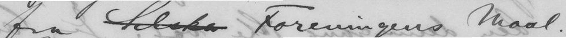fra Selska Foreningens Maal.
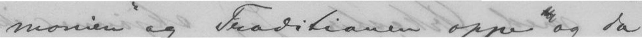monien" og Traditionen oppe og da
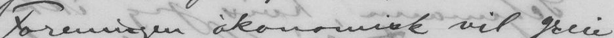Foreningen økonomisk vil greie
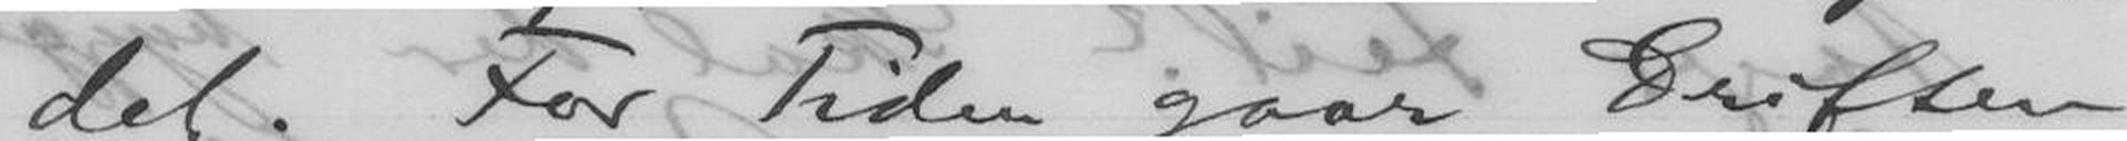det. For Tiden gaar Driften
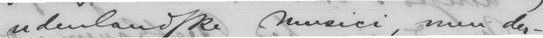udenlandske Musici, men der-
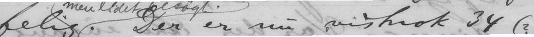felig. Der er nu vistnok 34 (?)
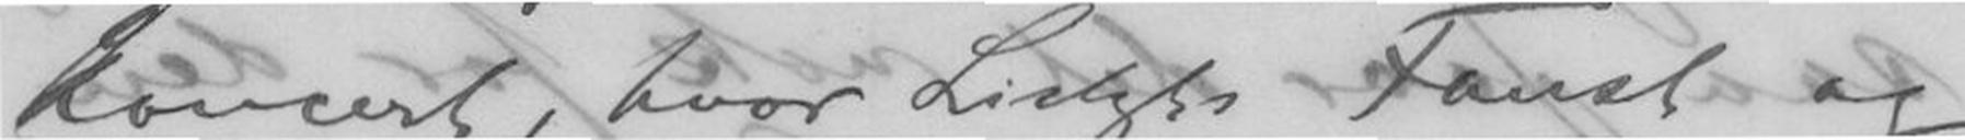koncert, hvor Listzs Faust og
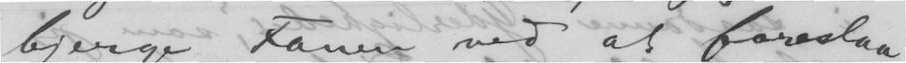bjerge Fanen ved at foreslaa
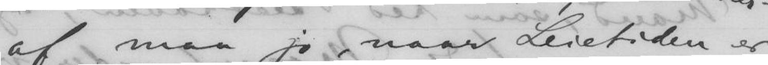af maa jo, naar Leietiden er
